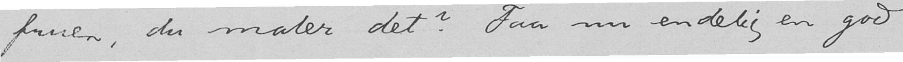fruen, du maler det? Faa nu endelig en god
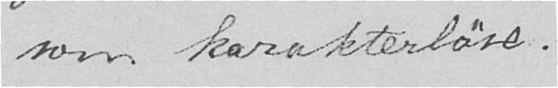som karakterløse.
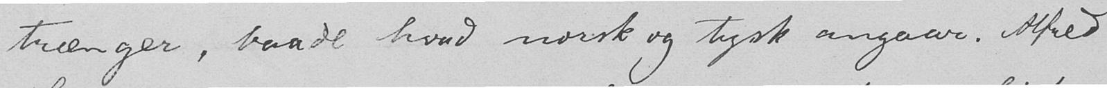trænger, baade hvad norsk og tysk angaar. Alfred
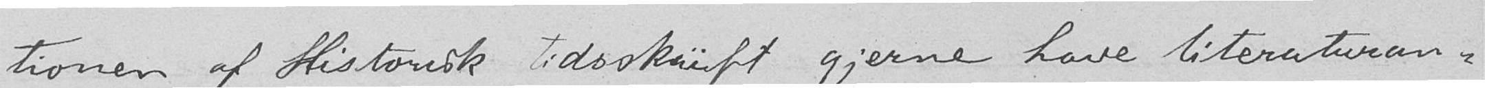tionen af Historisk tidsskrift gjerne have literaturan-
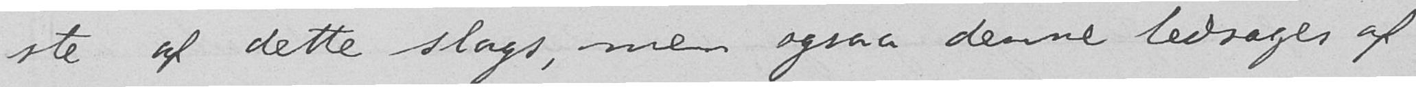ste af dette slags, men ogsaa denne ledsages af
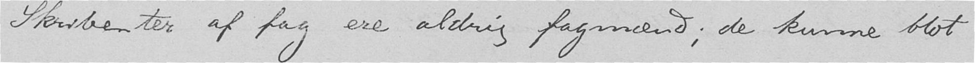Skribenter af fag ere aldrig fagmænd; de kunne blot
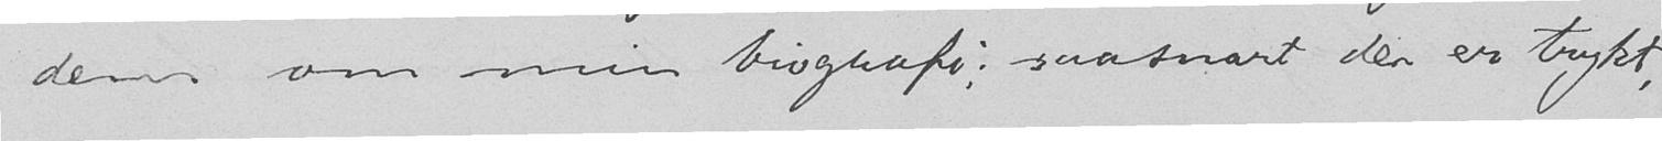dem om min biografi; saasnart den er trykt,
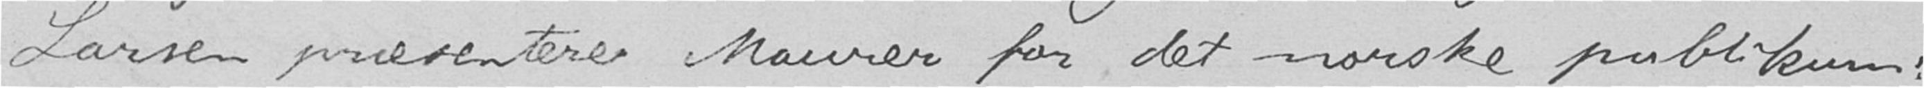Larsen præsenterer Maurer for det norske publikum.
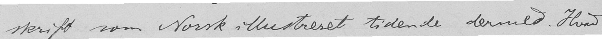skrift som Norsk illustreret tidende dermed. Hvad
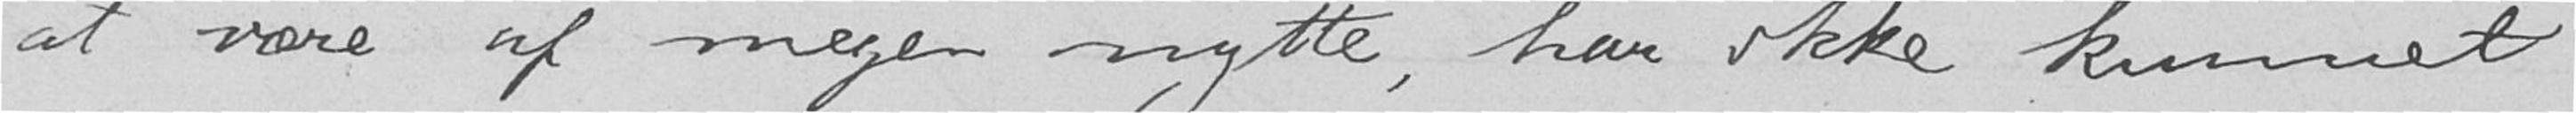at være af megen nytte, har ikke kunnet
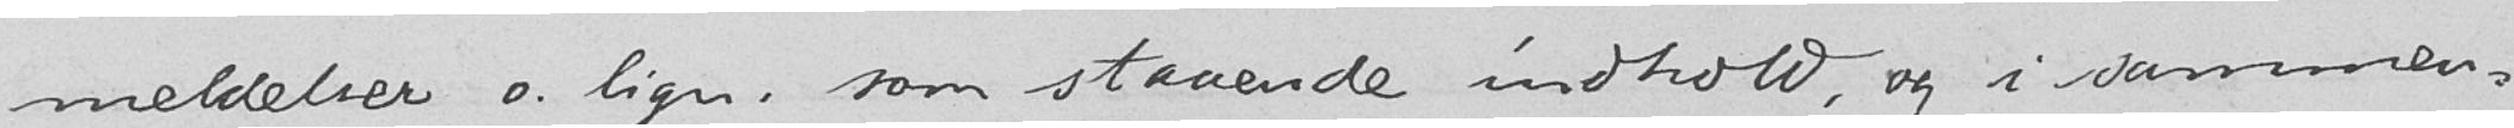meldelser o. lign. som staaende indhold, og i sammen-
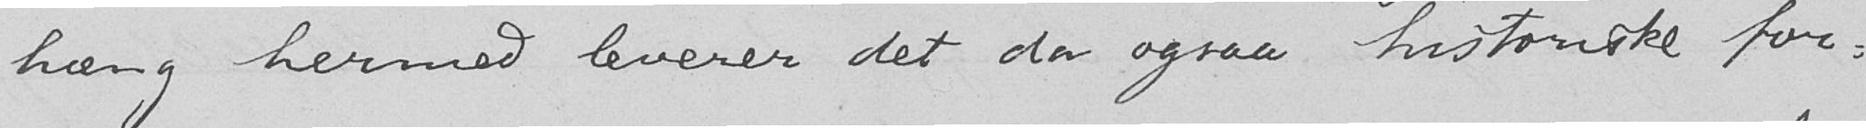hæng hermed leverer det da ogsaa historiske for-
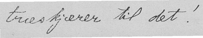træskjærer til det!
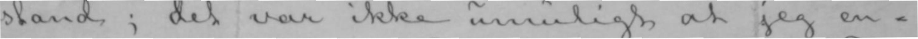stand; det var ikke umuligt at jeg en-
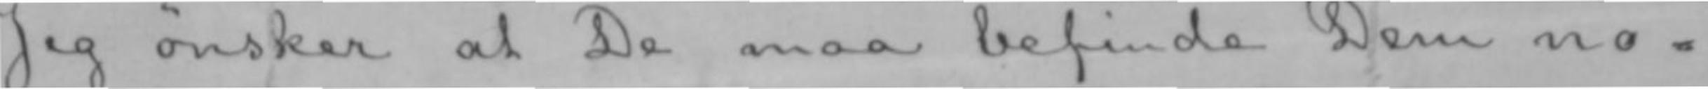Jeg ønsker at De maa befinde Dem no-
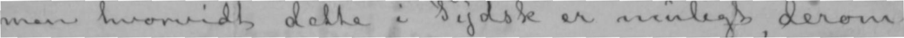men hvorvidt dette i Tydsk er muligt, derom
